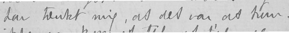har tænkt mig, at det var, at hun
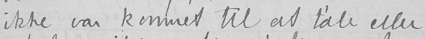ikke var kommet til at tale eller
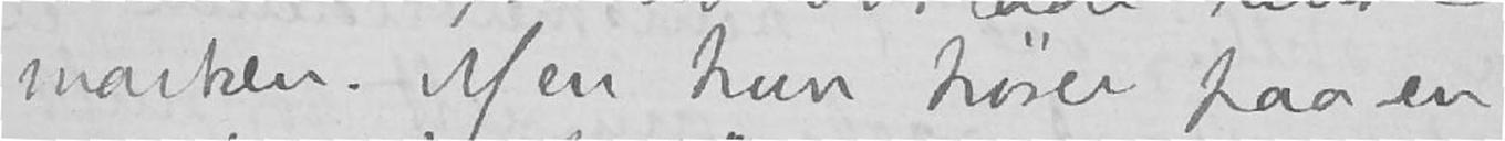marken. Men hun hører paa en
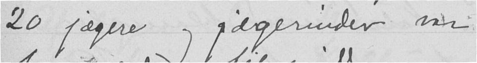20 jægere og jægerinder var
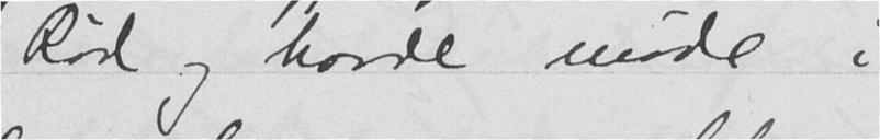Rød og havde møde i
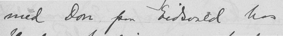med Don paa Eidsvold hos
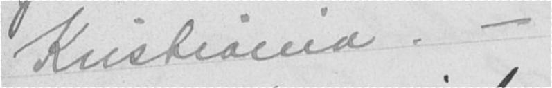Kristiania -
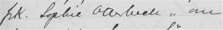frk. Sophie Otterbeck "om
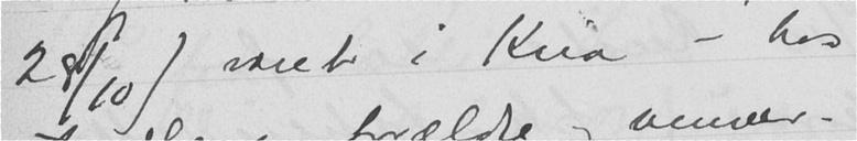28/10) været i Kria - hos
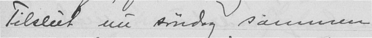Tilslut nu søndag sammen
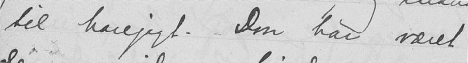til harejagt. Don har været
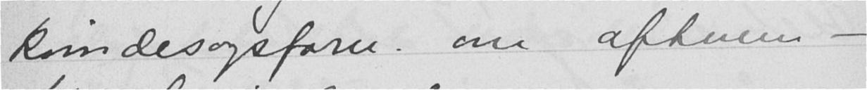kvindesagsforn. om aftenen -
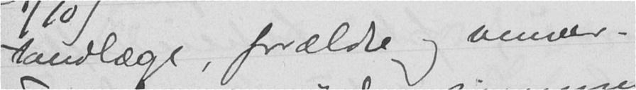tandlæge, forældre og venner.
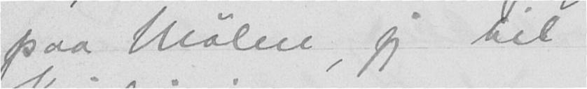paa Mølen, jeg til
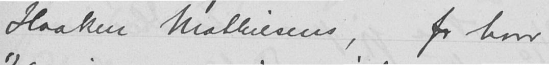Haaken Mathiesens, fo hvor
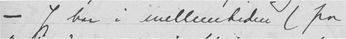- Jeg har i mellemtiden (fra
